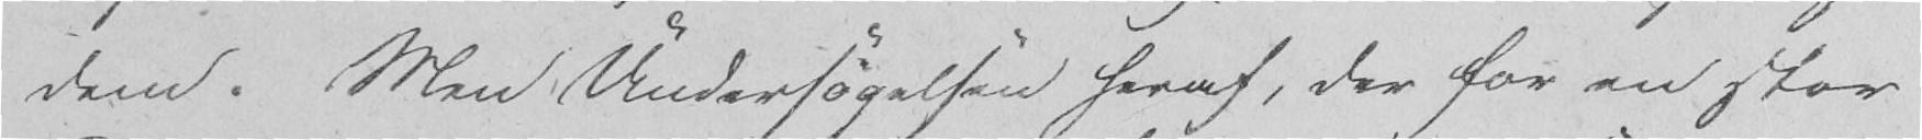dem. Men Undersøgelsen heraf, der for en stor
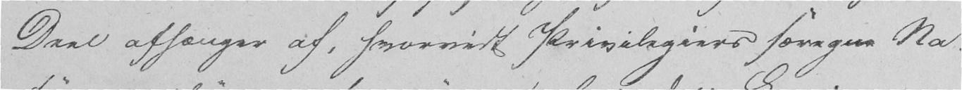Deel afhænger af, hvorvidt Privilegiers særegne Na-
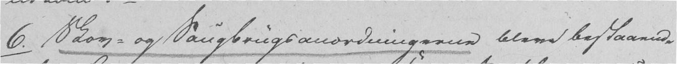6. Skov- og Saugbrugsanordningerne bleve bestaaende
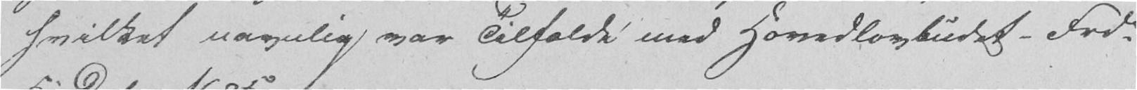hvilket navnlig var Tilfælde med Hovedlovbudet - Frdn
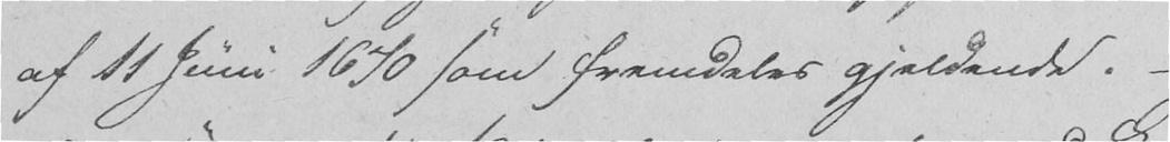af 11 Juni 1670 som fremdeles gjeldende. -
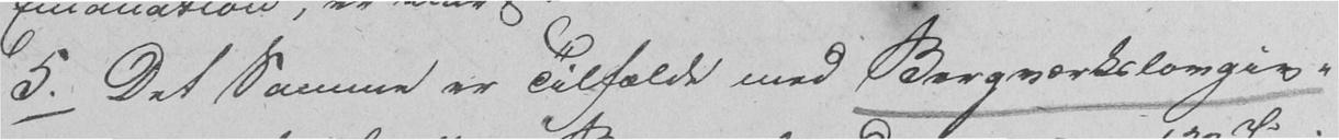5. Det Samme er Tilfælde med Bergværkslovgiv-
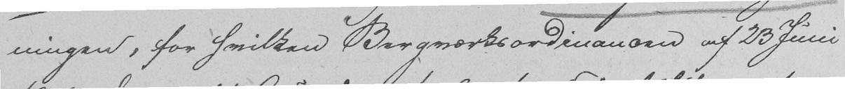ningen, for hvilken Bergværksordinancen af 23 Juni
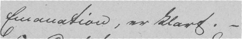Emanation, er klart. -
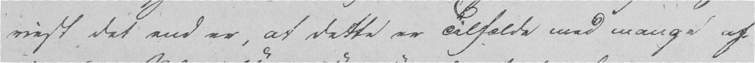vist det end er, at dette er Tilfælde med mange af
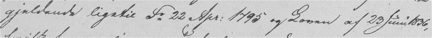gjeldende ligetil Fr 22 Apr. 1795 og Loven af 23 Juni 1836,
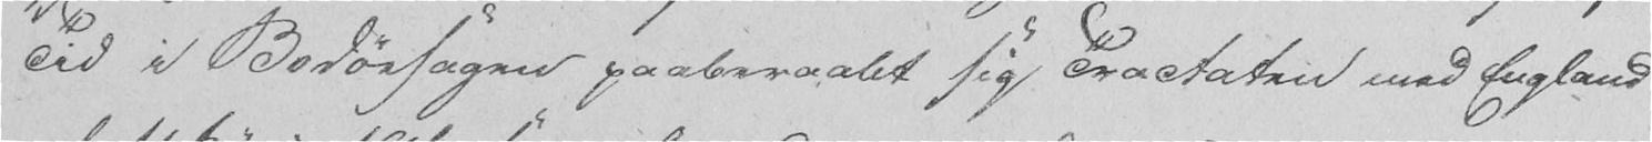Tid i Bodøesagen paaberaabt sig Tractaten med England
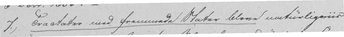7. Tractater med fremmede Stater bleve naturligviis
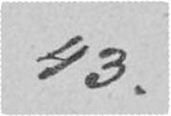43.
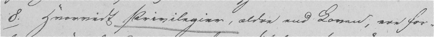8. Hvorvidt Privilegier, ældre end Loven, ere for-
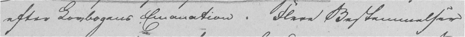efter Lovbogens Emanation. Flere Bestemmelser
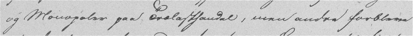og Monopoler paa Trælasthandel, men andre forbleve
